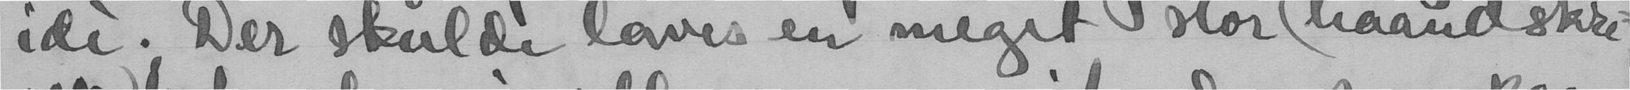ide. Der skulde laves en meget stor (haandskre-
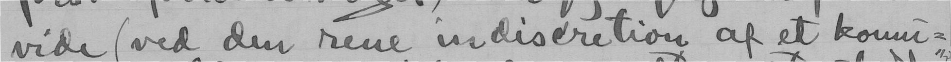vide (ved den rene indiseretion af et komu-
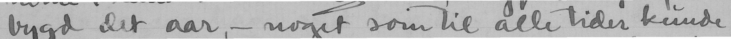bygd det aar - noget som til alle tider kunde
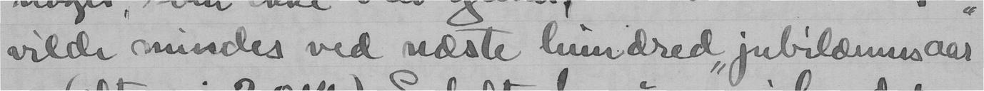vilde mindes ved næste hundred "jubilæumsaar"
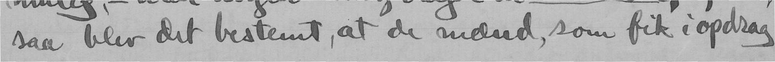saa blev det bestemt, at de mænd, som fik i opdrag
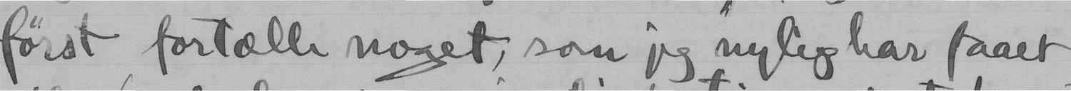først fortælle noget, som jeg nyleg har faaet
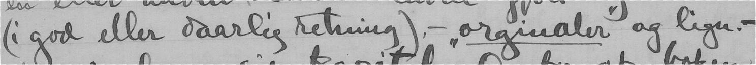(i god eller daarlig retning), - "orginaler" og lign.-
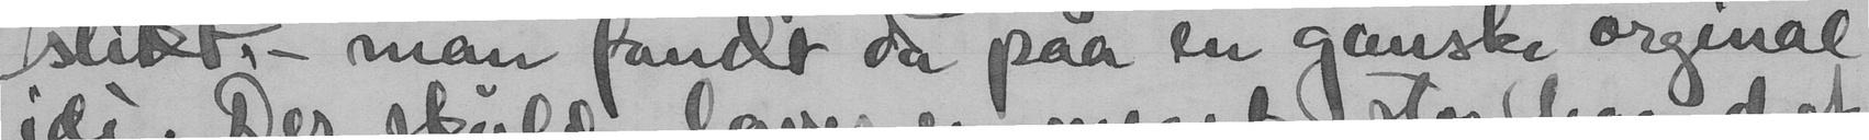slikt, - man fandt da paa en ganske orginal
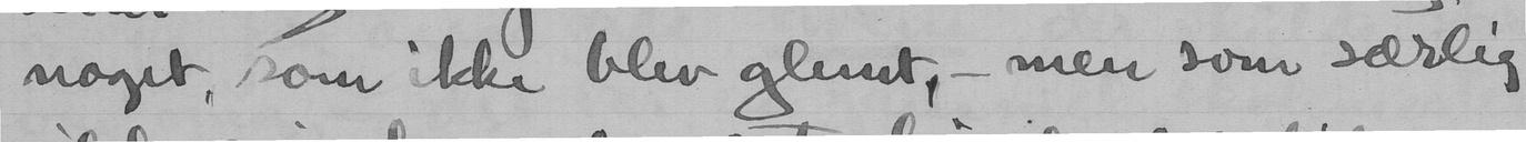noget som ikke blev glemt, - men som særlig
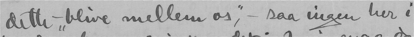dette - blive mellem os", - saa ingen her i
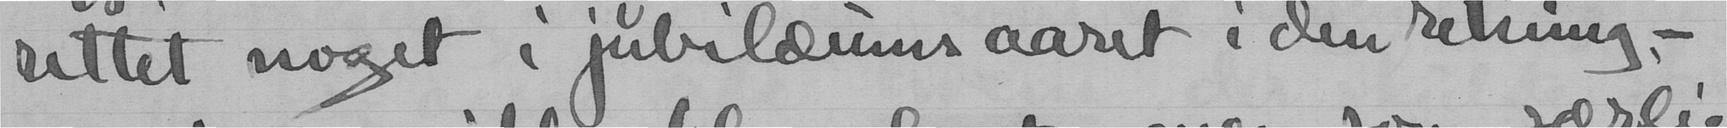rettet noget i jubilæums aaret i den retning,-
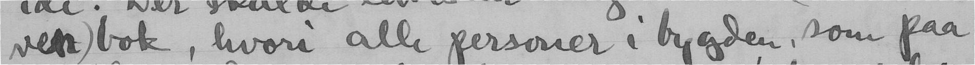ven) bok, hvori alle personer i bygden, som paa
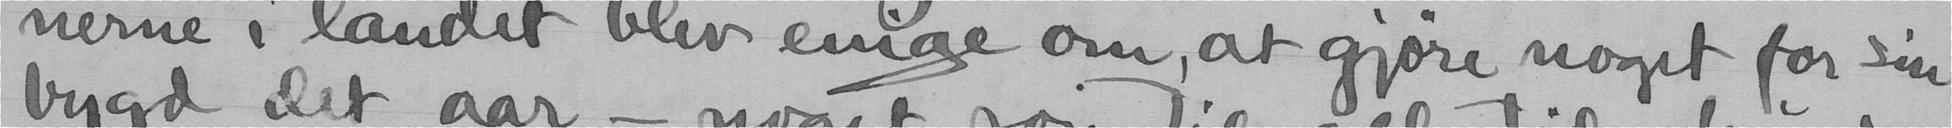nerne i landet blev enige om, at gjøre noget for sin
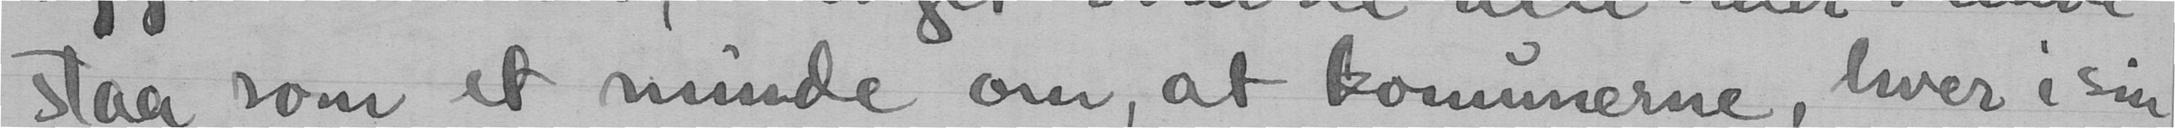staa som et minde om, at komunerne, hver i sin
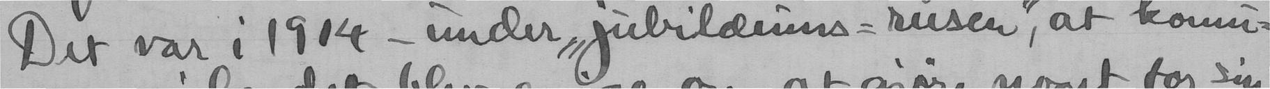Det var i 1914 - under "jubilæums-reisen", at komu-
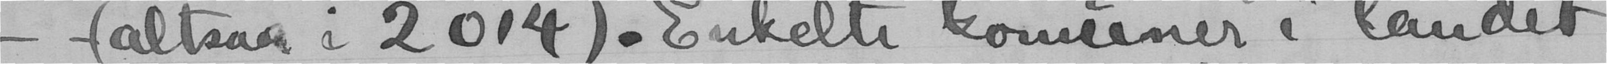- - (altsaa i 2004). Enkelte komuner i landet
